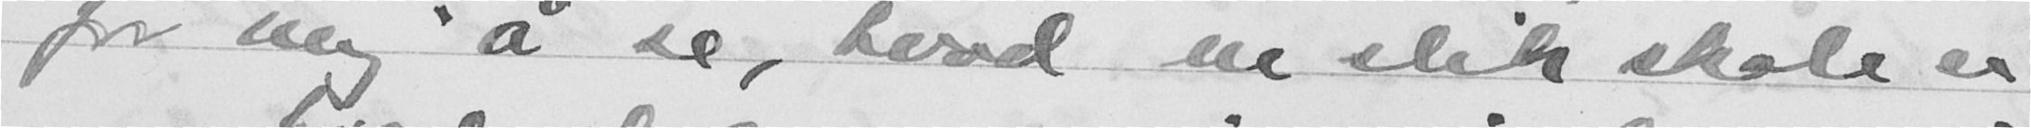for mig å se, hvad en slik skole er
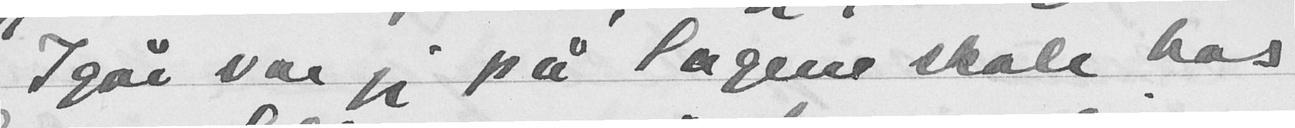Igår var jeg på Sagene skole hos
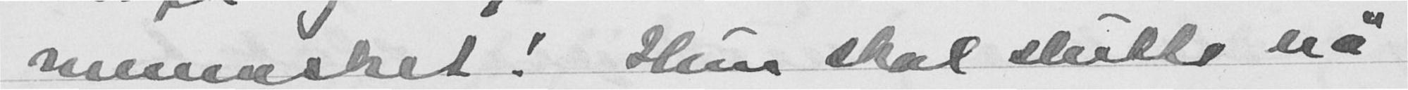mennesket! Hun skal slutte nå,
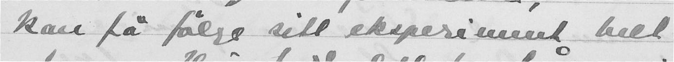kan få følge sitt eksperiment helt
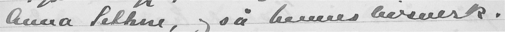Anna Sethne, og så hennes livsverk.
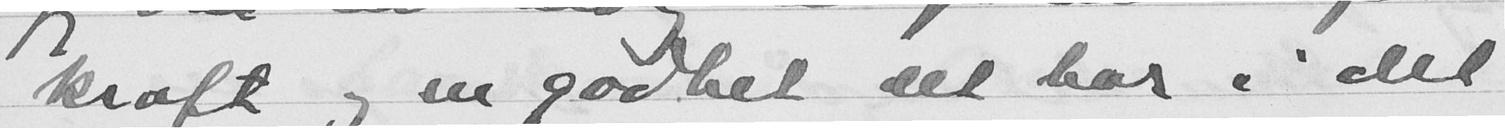kraft og en godhet det bor i det
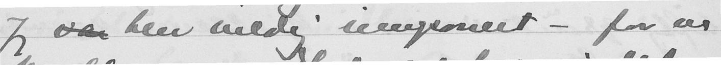Jeg  blev veldig imponert - for en
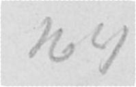109
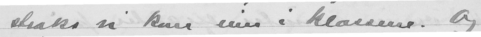straks vi kom inn i klassene. Og
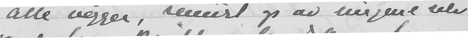alle vegger, smurt op av ungene selv
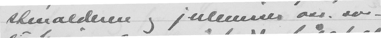steinalderen og julenisser oss. sv -
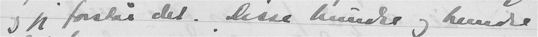og jeg forstår det. Disse hundre og hundre
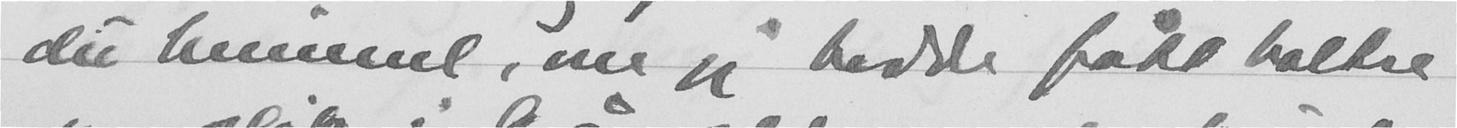du himmel, om jeg hadde fått boltre
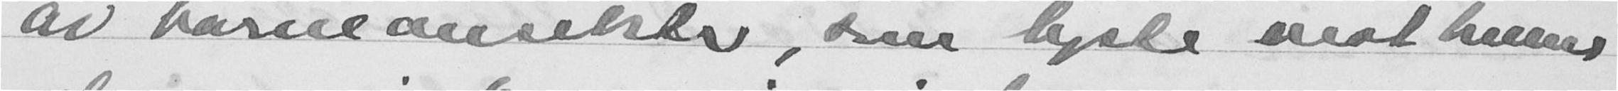av barneansikter, som lyste mot henne
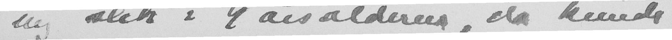mig slik i 9 årsalderen, da kunde
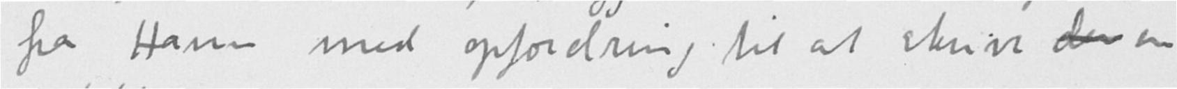fra Hann med opfordring til at skrive der en
